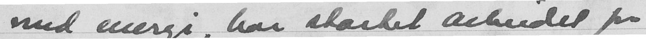med energi, har startet arbeidet for
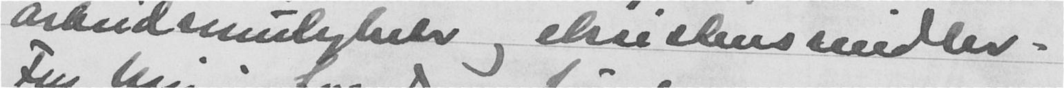arbeidsmuligheter og eksistensmidler.
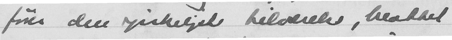fører den sykeligste tilværelse, blottet
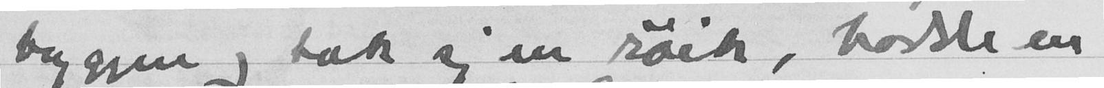bryggen og tok sig en røyk, hadde en
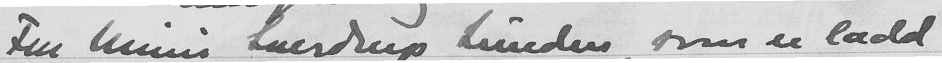Fru Mimi Sverdrup Lunden, som er ladd
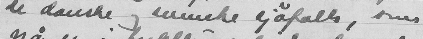de danske og svenske sjøfolk, som
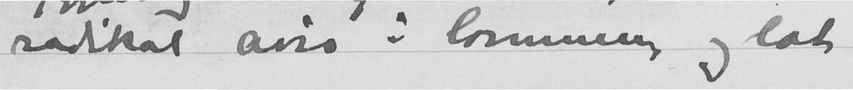radikal avis i lommen og lot
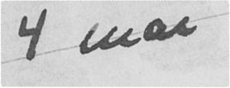4 mai
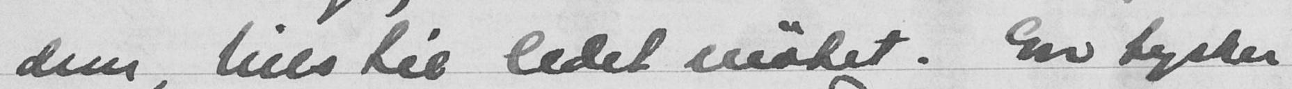dem, Nils Lie ledet møtet. En tysker
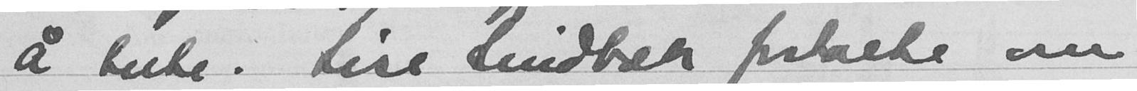å tute. Lise Lindbæk fortalte om
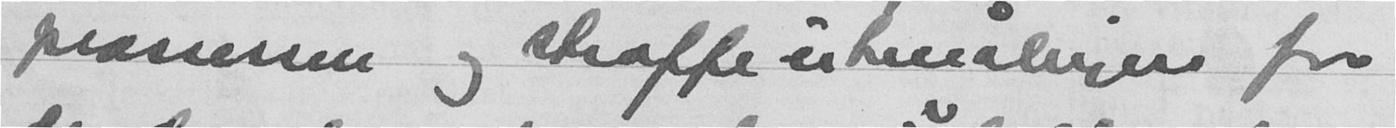prossessen og straffeutmålingen for
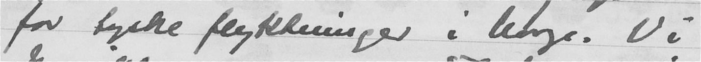for tyske flyktninger i Norge. Vi
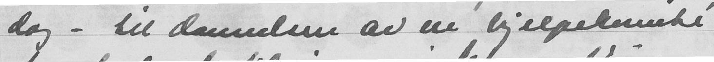dag - til dannelsen av en hjelpekomité
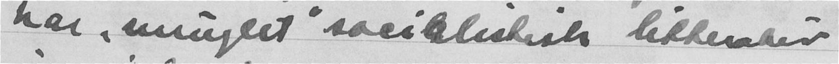har "smuglet" socialistisk litteratur
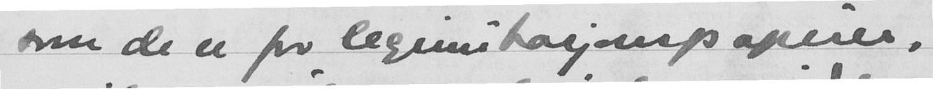som de er for legimitasjonspapirer,
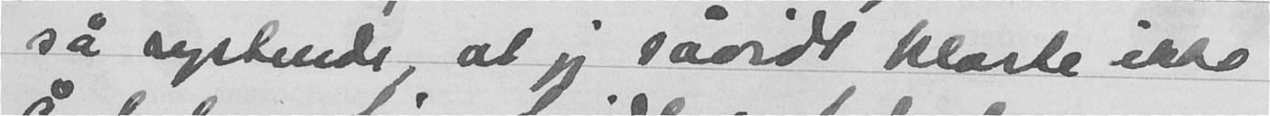så rystende, at jeg såvidt klarte ikke
